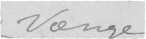Vænge.
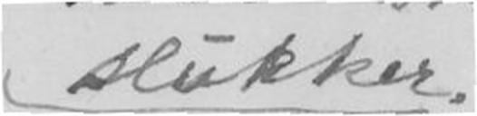slukker.
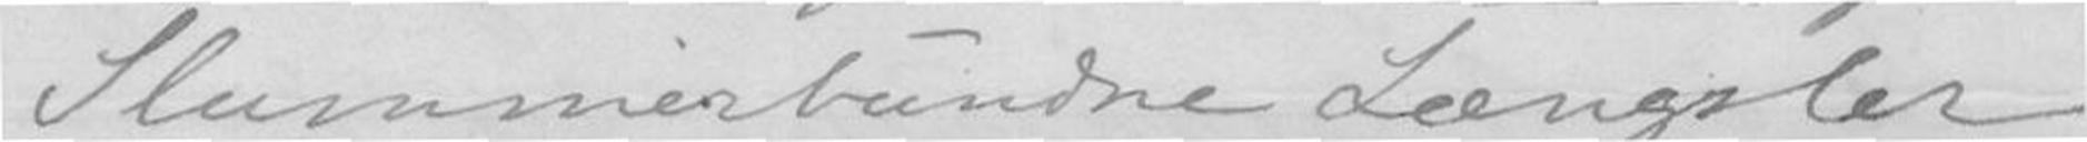Slummerbundne Længsler
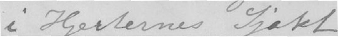i Hjerternes Sjakt
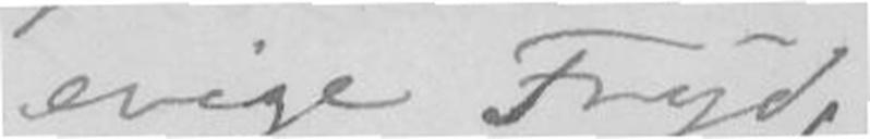evige Fryd,
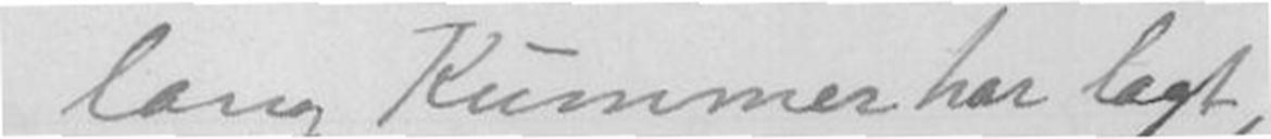lang Kummer har lagt,
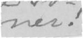ner!
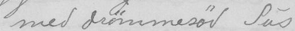med drømmesød Sus
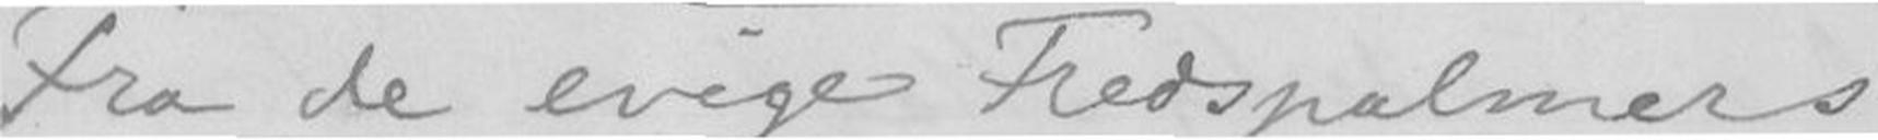Fra de evige Fredspalmers
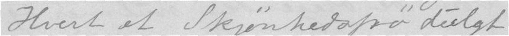Hvert et Skjønhedsfrø dulgt
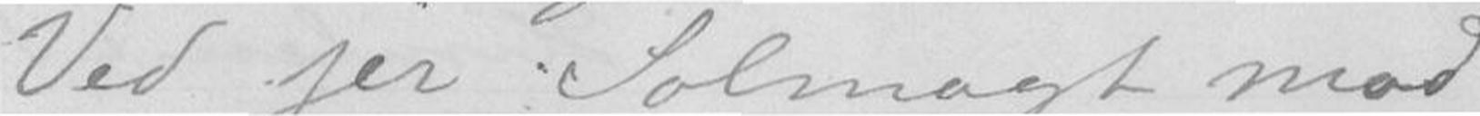Ved jeg Solmagt mod
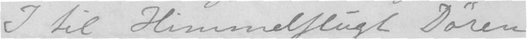I til Himmelflugt Døren
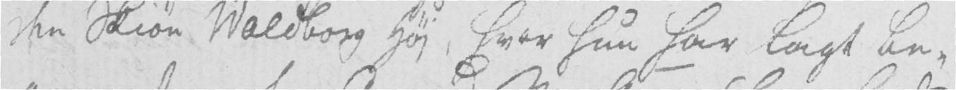den Skiøn Waldborg Høj, hvor hun har lagt be-
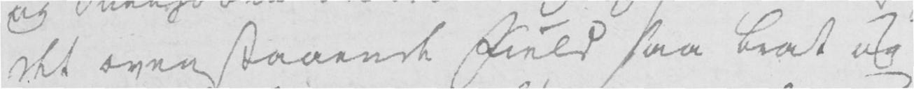det ovenstaaende Field saa brat og
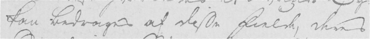kan bedrages af disse Fielde, deres
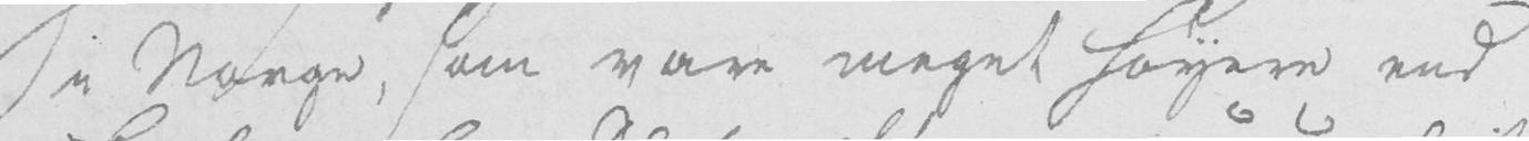i Norge, som vare meget høyere end
